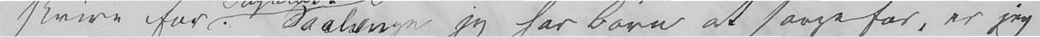skrive for Saalænge jeg har Børn at sørge for, er jeg
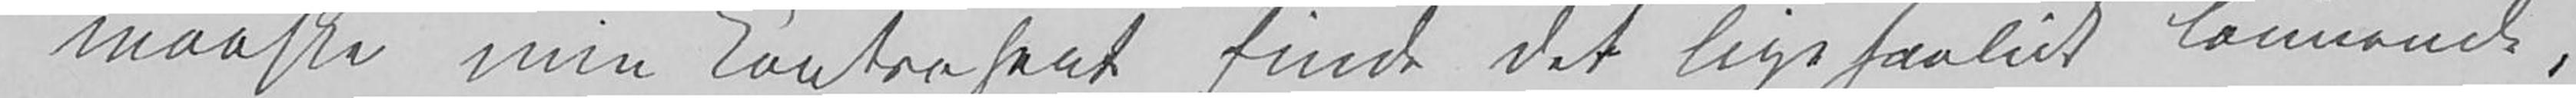maaske min Kontrahent finde det ligesaalidt lønnende,
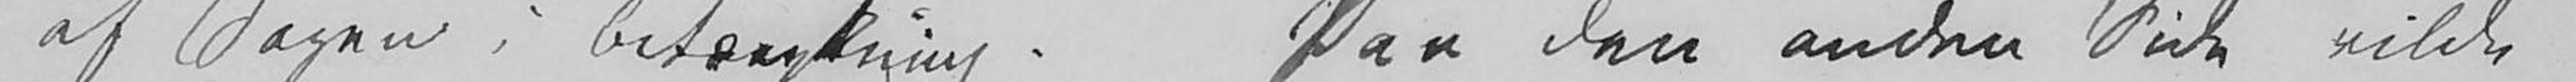af Sagen i Betænkning. Paa den anden Side vilde
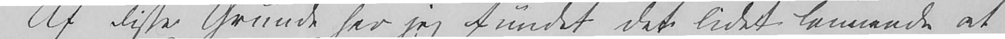Af disse Grunde har jeg fundet det lidet lønnende at
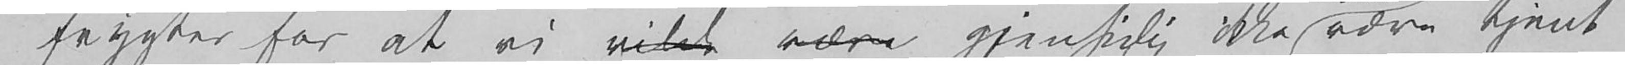frygter for at vi vilde være gjensidig ikke være tjent
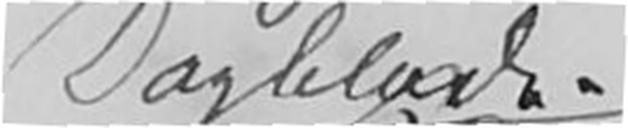Dagblade.
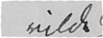vilde
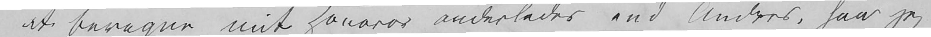at beregne mit Honorar anderledes end Andres, saa jeg
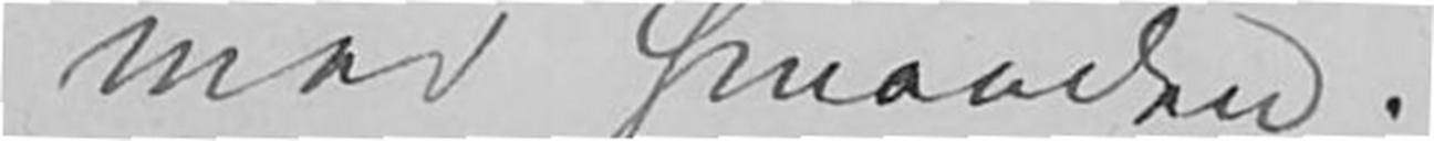med hinanden.
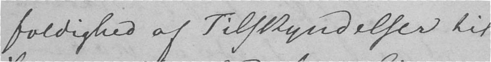foldighed af Tilskyndelser til
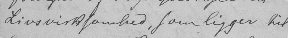Livsvirksomhed, som ligger til
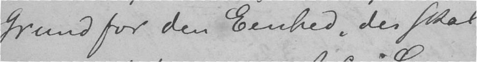Grund for den Eenhed, der skal
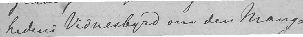hedens Vidnesbyrd om den Mang-
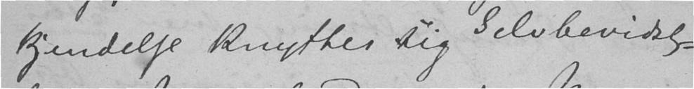kjendelse knytter sig Selvbevidst-
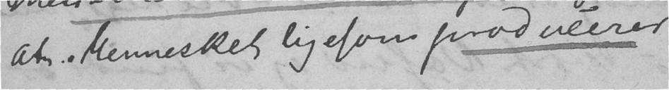at Mennesket ligesom producerer
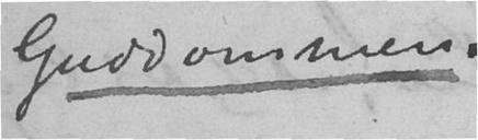Guddommen.
